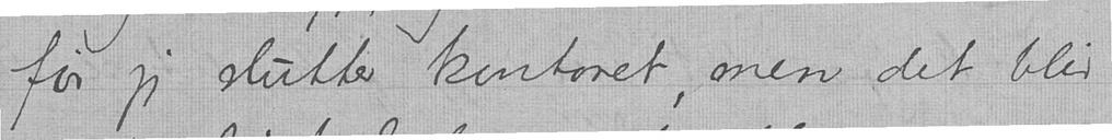før jeg slutter kontoret, men det blir
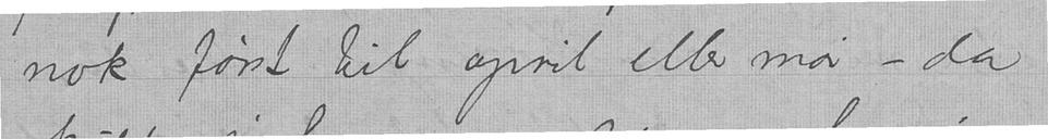nok først til april eller mai — da
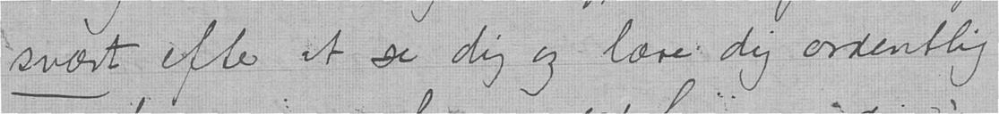svært efter at se dig og lære dig ordentlig
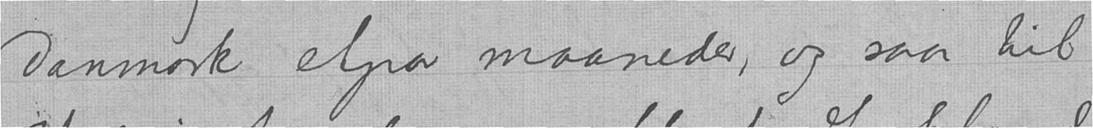Danmark etpar maaneder, og saa til
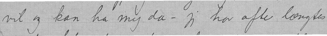vil og kan ha mig da — jeg har ofte længtes
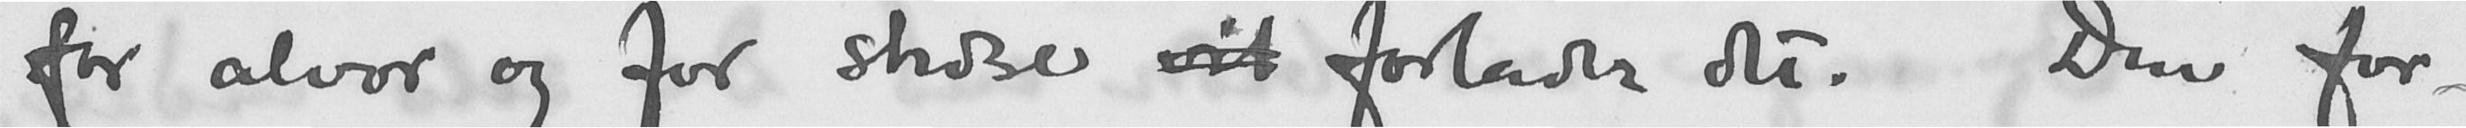for alvor og for stedse vil forlader det. Den for-
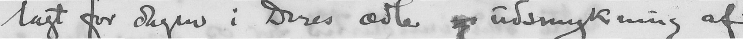lagt for dagen i Deres ædle y udsmykning af
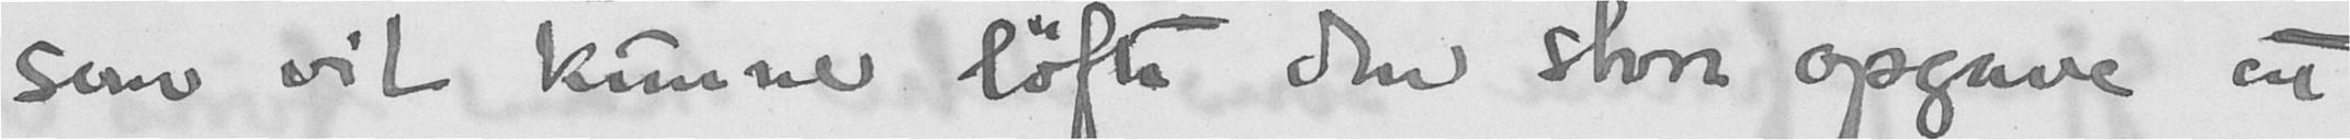som vil kunne løfte den store opgave at
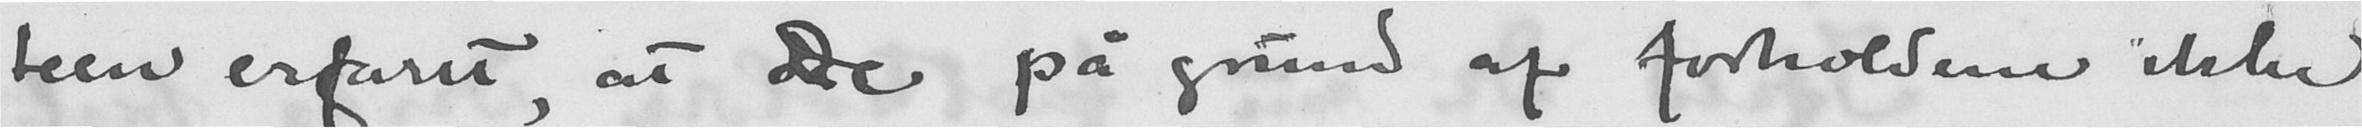teen erfaret, at der på grund af forholdene ikke
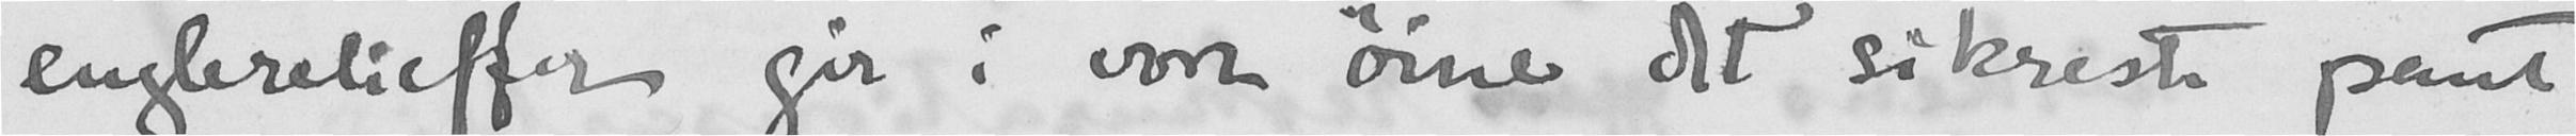englerelieffer gir i vore øine det sikreste pant
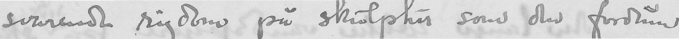svarende rigdom på skulptur som den fordum
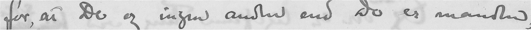for, at De og ingen anden end De er manden,
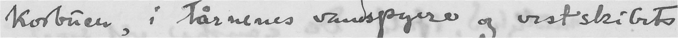korbuen, i tårnenes vandspyere og vestskibets
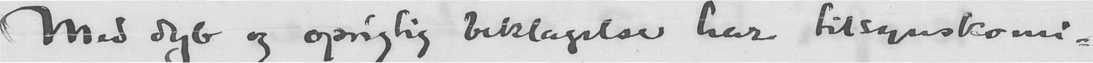Med dyb og oprigtig beklagelse har tilsynskomi-
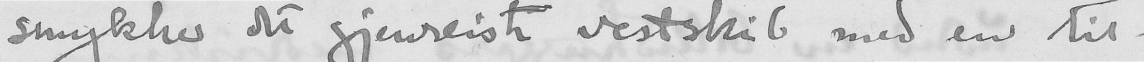smykke det gjenreiste vestskib med en til-
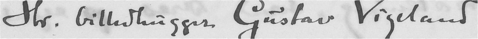Hr. billedhugger Gustav Vigeland
